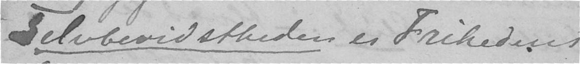Selvbevidstheden er Frihedens
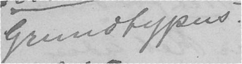Grundtypus.
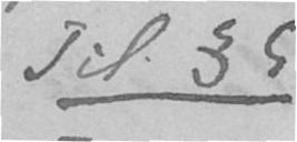Til § 5
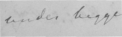under begge
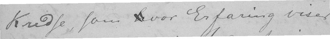Kredse, som hvor Erfaring viser
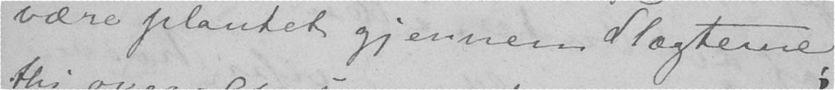være plantet gjennem Slægterne;
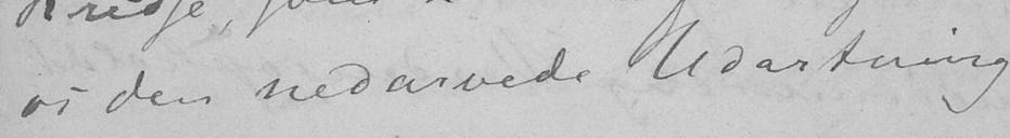os den nedarvede Udartning
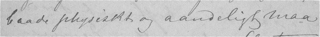baade physiskt og aandeligt maa
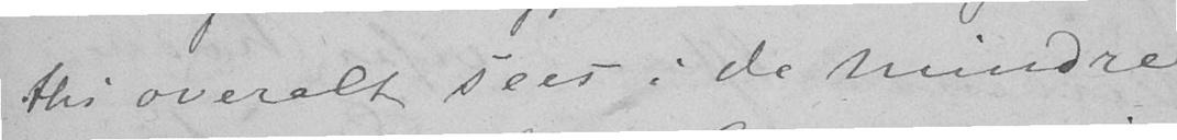thi overalt sees i de mindre
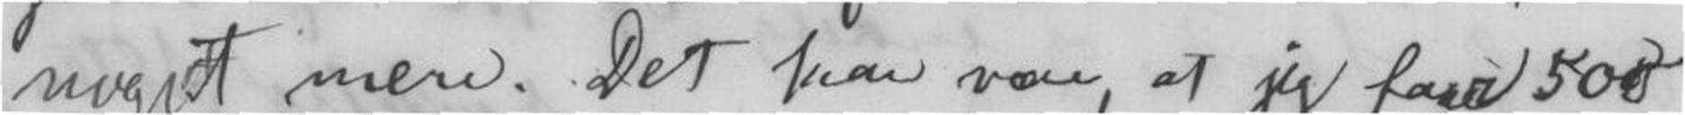noget mere. Det kan være, at jeg faar 500
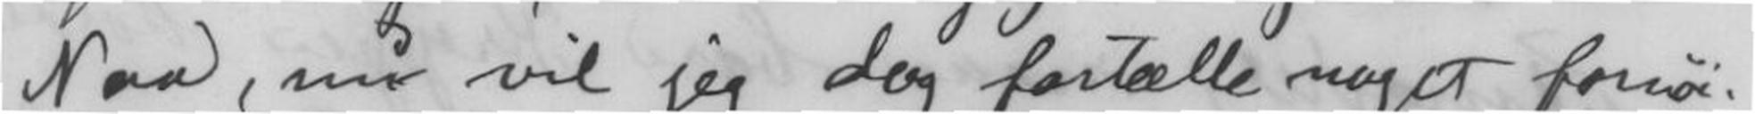Naa, nu vil jeg dog fortælle noget fornøi-
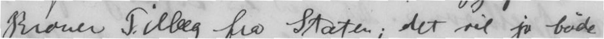Kroner Tillæg fra Staten; det vil jo bøde
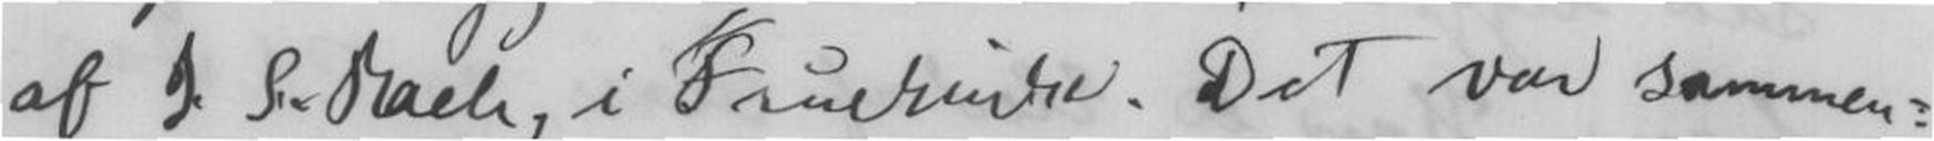af I.S.Bach, i Fruekirke. Det var sammen-
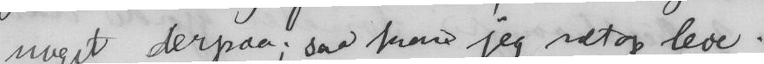meget derpaa; saa kan jeg netop leve.
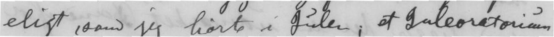eligt, som jeg hørte i Julen; et Juleoratorium
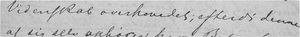Videnskab overhovedet; efterdi denne
